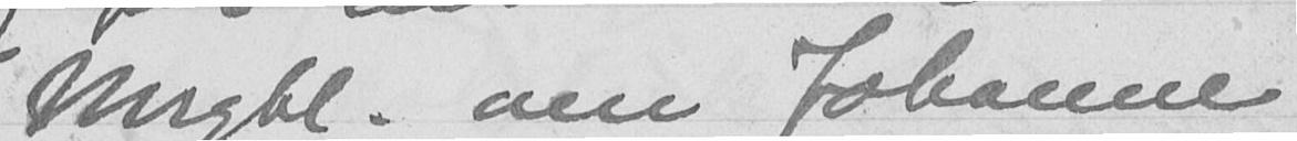Mrgbl. om Johanne
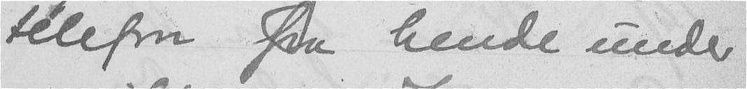telefon fra hende under
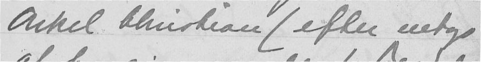Onkel Christian (efter netop
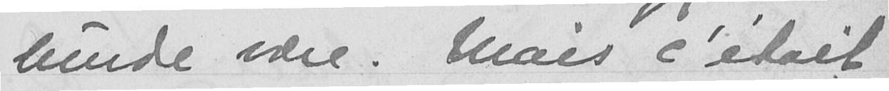burde være. Mais c'était
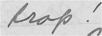trop!
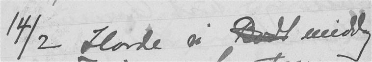14/2 Havde vi Bodt middag
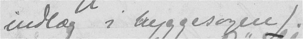indlæg i byggesagen).
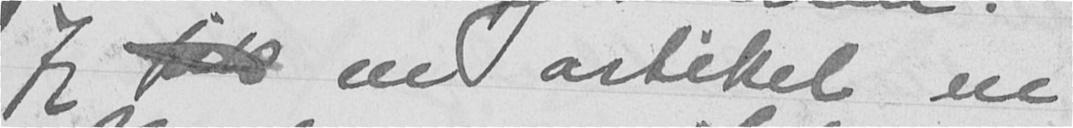Jeg fik en artikel en
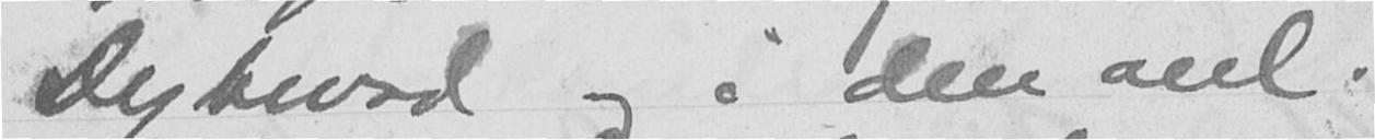Dybwad og i den anl.
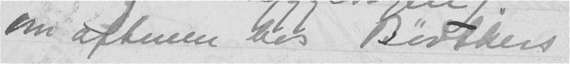Om aftenen hos Bødtkers
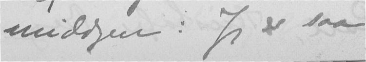middagen: Jeg er saa
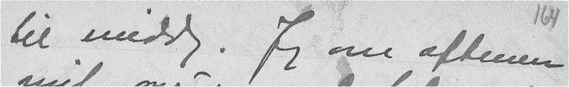til middag. Jeg om aftenen
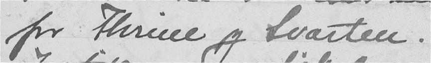for Thrine og Svarten.
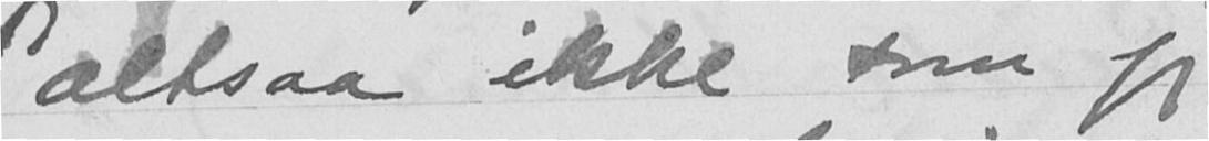altsaa ikke som jeg
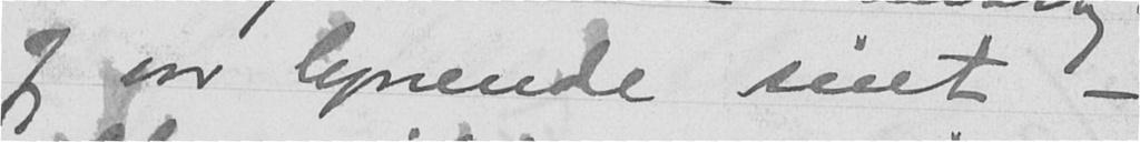Jeg var lynende sint -
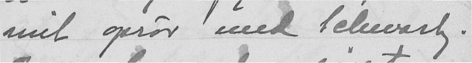mit oprør med Schwartz.
